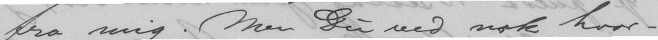fra mig. Men Du ved nok hvor-
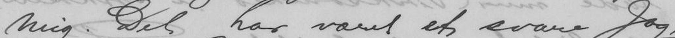mig. Det har været et svare Jag,
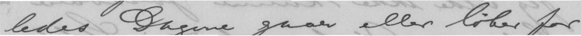ledes Dagene gaar eller løber for
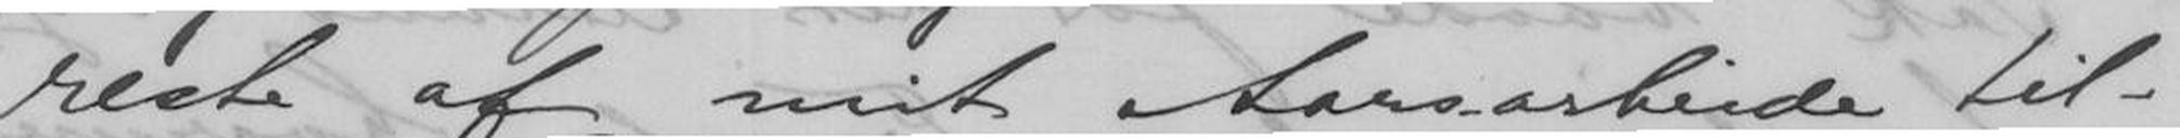reste af mit Aarsarbide til-
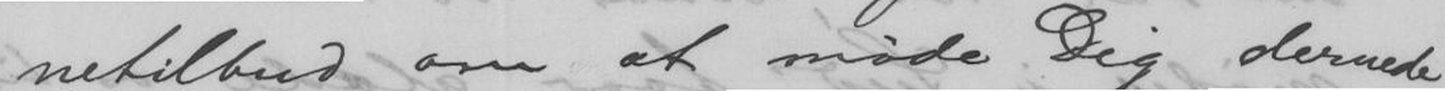netilbud om at møde Dig dernede,
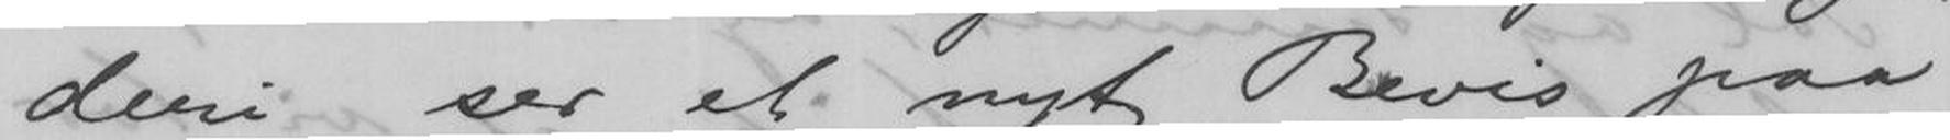deri ser et nyt Bevis paa
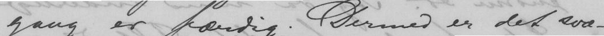gang er færdig. Dermed er det svæ-
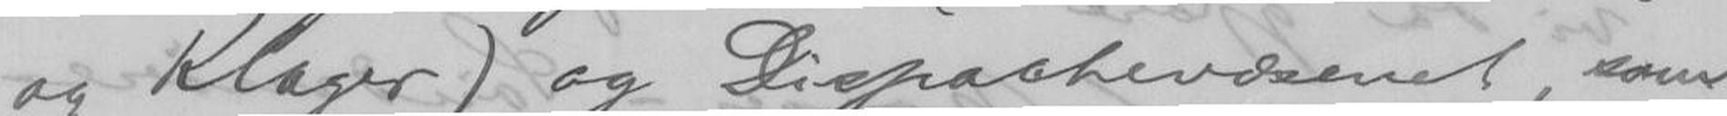og Klager) og Dispachevæsenet, som
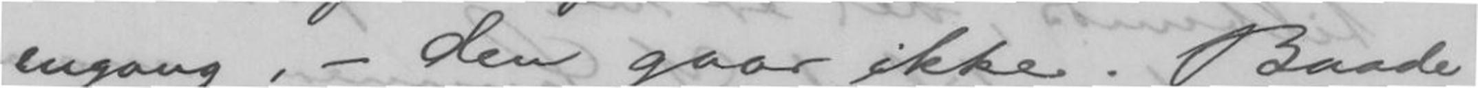engang, - den gaar ikke. Baade
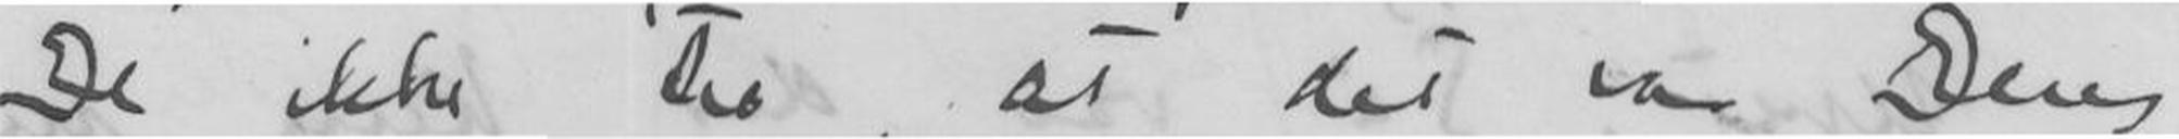De ikke tro, at det var Deres
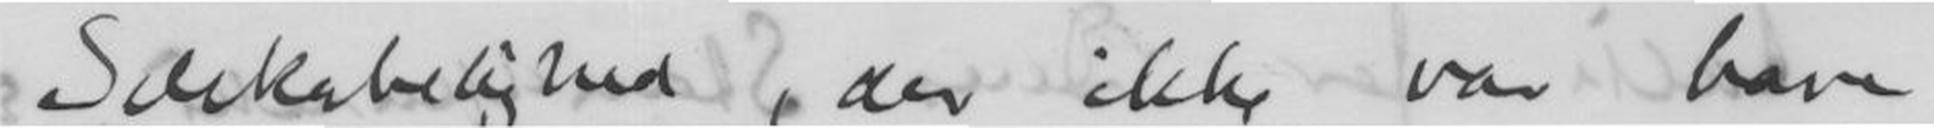Selskabelighed, der ikke var bare
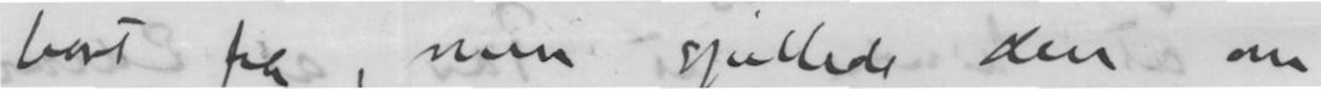bort fra, men spillede den om
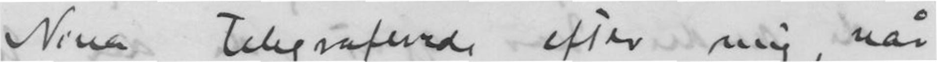Nina telegraferede efter mig, når
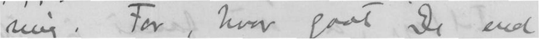mig, For, hvor godt De end
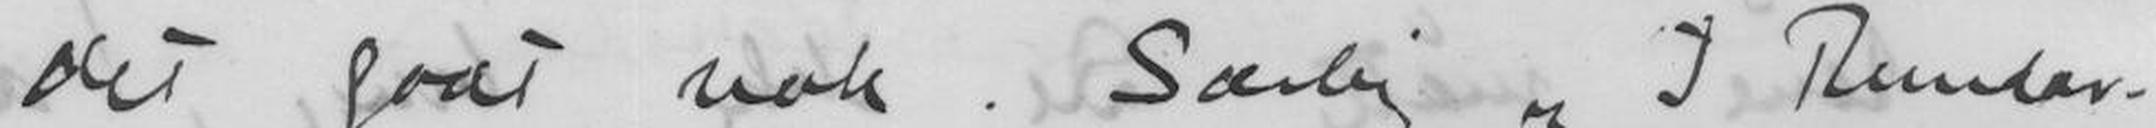det godt nok. Særlig I Rundar-
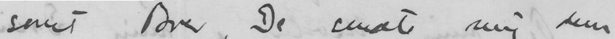somt Brev, De sendte mig dem
